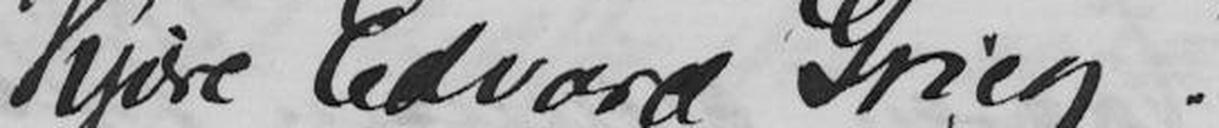Kjære Edvard Grieg!
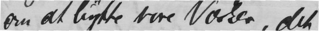om at bytte vore Værker, det
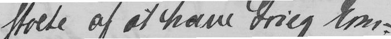stolte af at have Grieg Com-
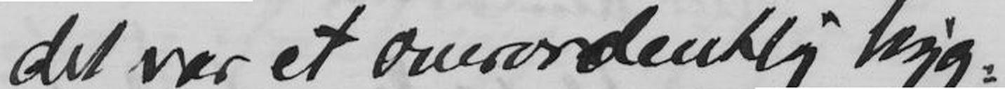det var et overordentlig hyg-
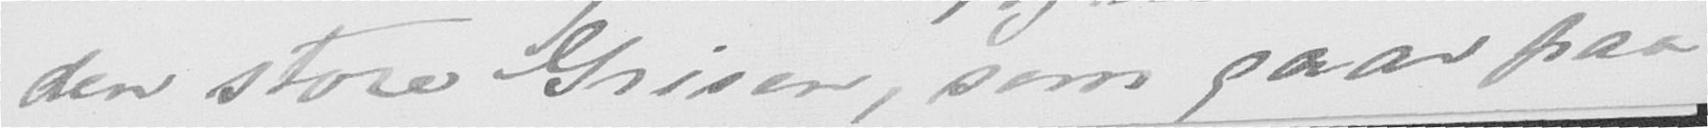den store Grisen, som gaar paa
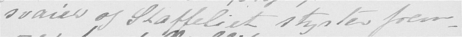svaier og Staffeliet styrter frem-
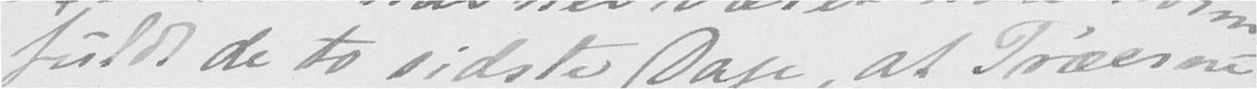fuldt de to sidste Dage, at Træerne
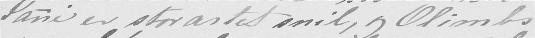Janni er storartet snil, og Olimbs
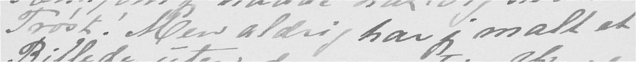Trost! Men aldrig har jeg malt et
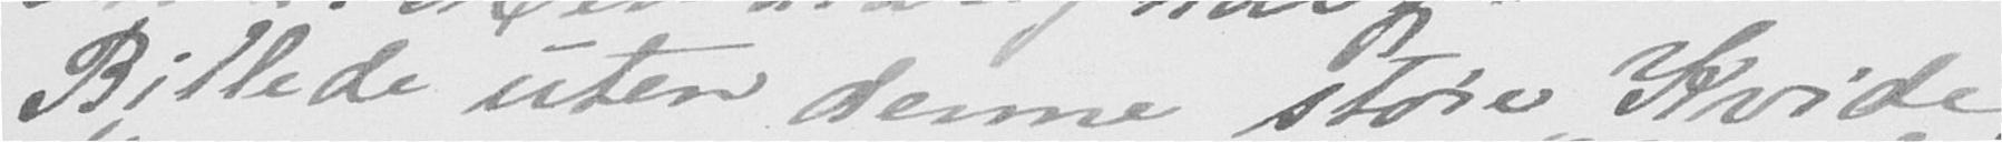Billede uten denne store Kvide.
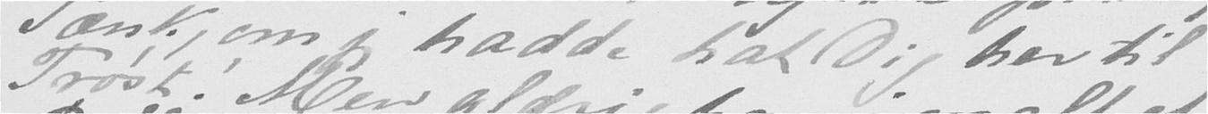Tænk, om jeg hadde hat Dig her til
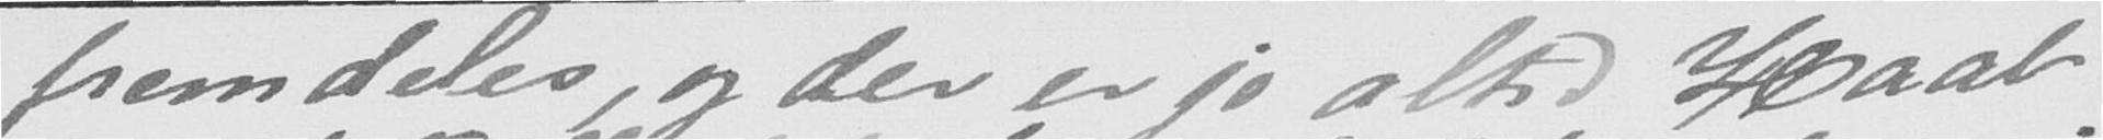fremdeles, og der er jo altid Haab
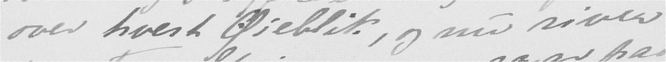over hvert Øieblik, og nu river
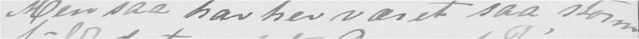Men saa har her været saa storm-
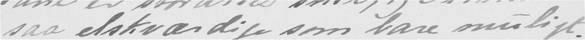saa elskværdige som bare muligt.
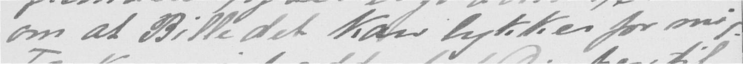om at Billedet kan lykkes for mig.
